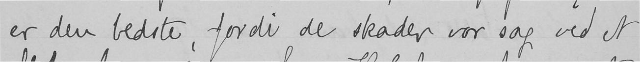er den bedste, fordi de skader vor sag ved et
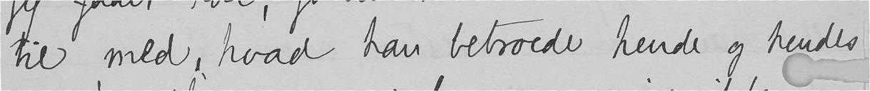tie med, hvad han betroede hende og hendes
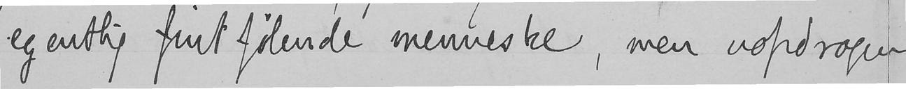egentlig fint følende menneske, men uopdragen
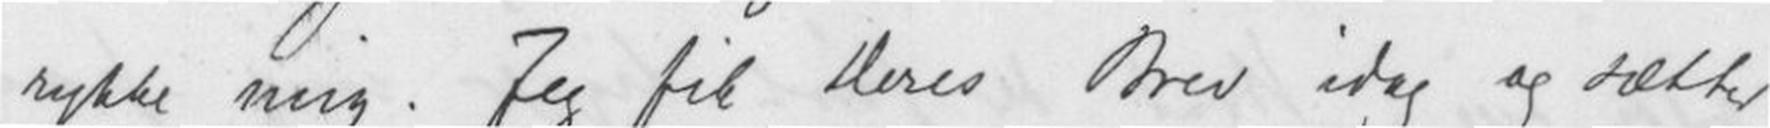rykke mig. Jeg fik Deres Brev idag og sætter
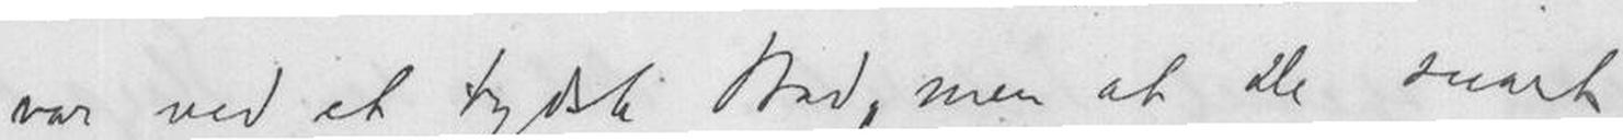var ved et tysk Bad, men at De snart
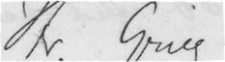Hr. Grieg!
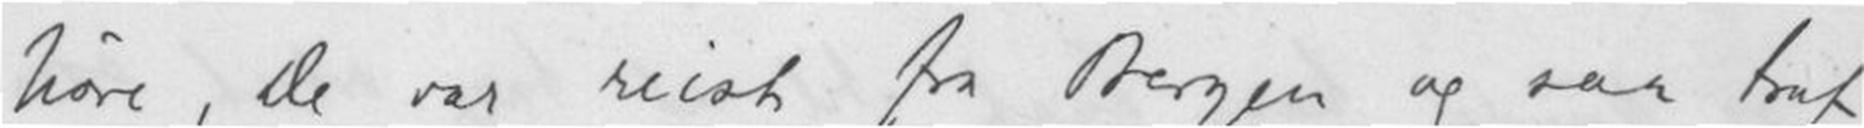høre, De var reist fra Bergen og saa traf
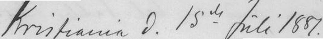Kristiania d. 15de Juli 1881
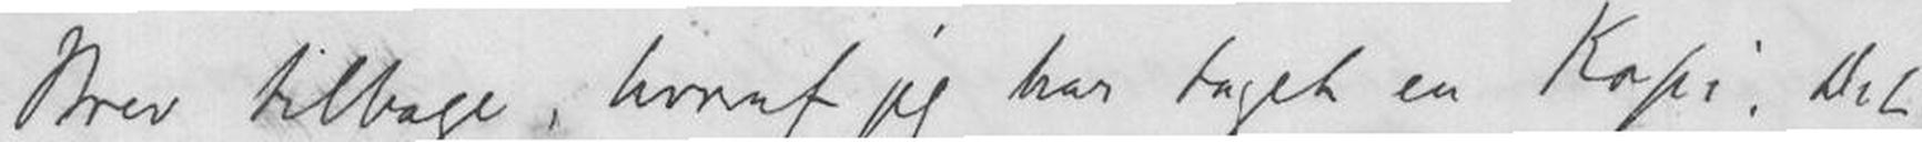Brev tilbage hvoraf jeg har taget en Kopi: Det
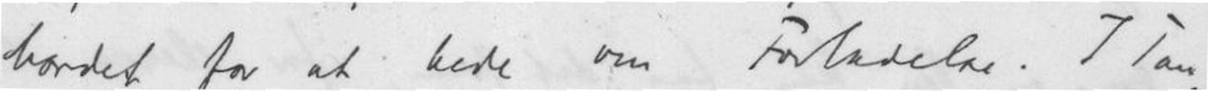bordet for at bede om Forladelse. I Tan-
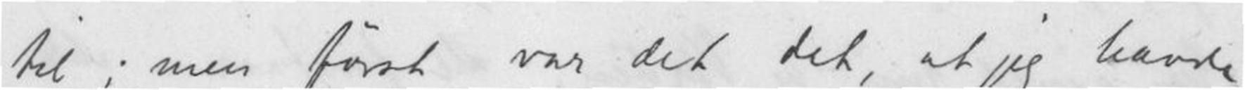til ; men først var det det, at jeg havde
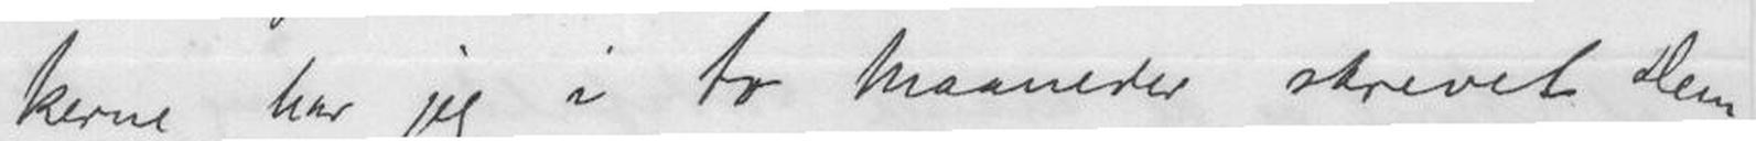kerne har jeg i to Maaneder skrevet Dem
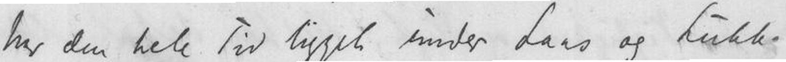har den hele tid ligget under Laas og Lukk
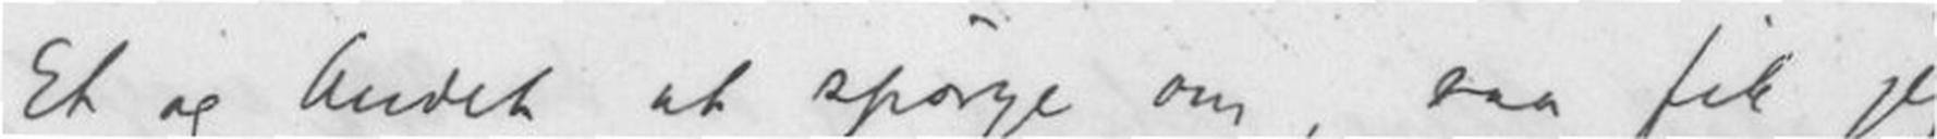Et og Andet at spørge om, saa fik jeg
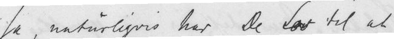Ja, naturligvis har De Lov til at
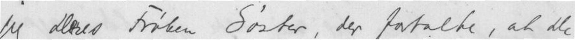jeg Deres Frøken Søster, der fortalte, at De
